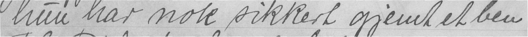hun har nok sikkert gjemt et ben
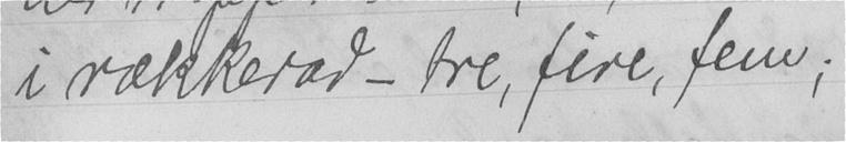i rækkerad - tre, fire, fem;
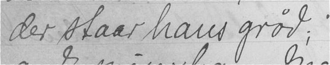der staar hans grød;
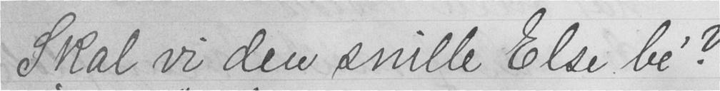Skal vi den snille Else be'?
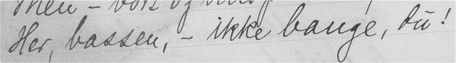Her, bassen, - ikke bange, du!
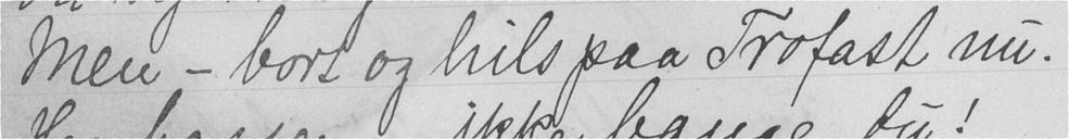Men - bort og hils paa Trofast nu.
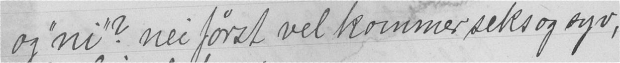og "ni"? nei først vel kommer seks og syv,
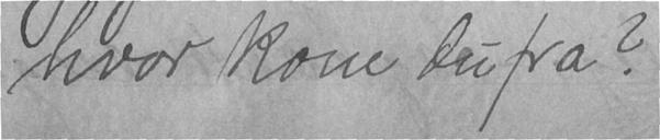hvor kom du fra?
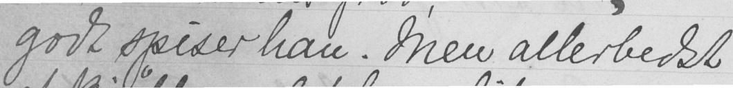godt spiser han. Men allerbedst
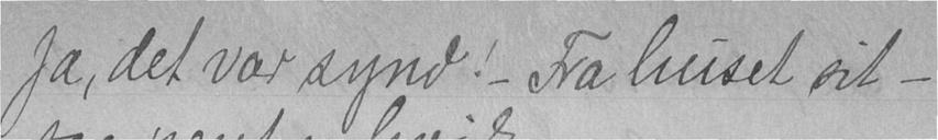Ja, det var synd! - Fra huset sit -
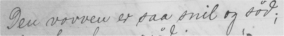Den vovven er saa snil og sød;
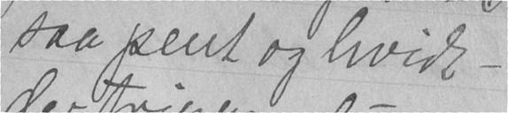saa pent og hvidt -
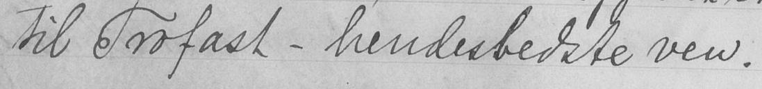til Trofast - hendesbedste ven.
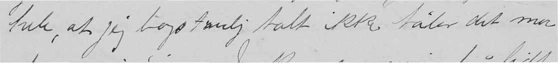hele, at jeg bogstavlig talt ikke tåler det mer
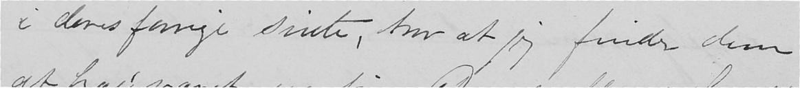i deres forrige sinte, tror at jeg finder dem
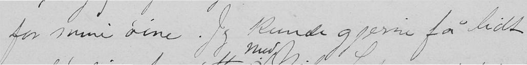for mine øine. Jeg kunde gjerne få lidt
